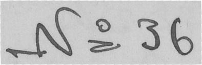No 36
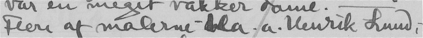Flere af malerne - bla.a. a. Henrik Lund, -
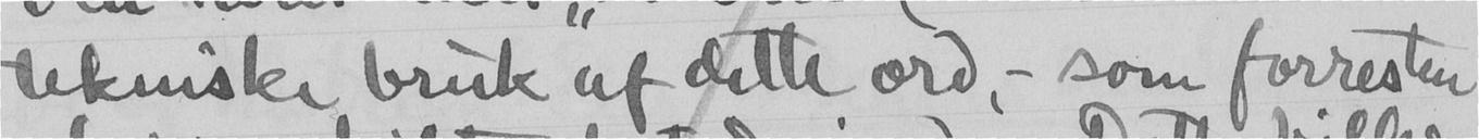tekniske bruk af dette ord - som forresten
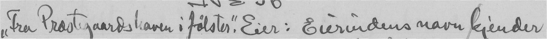"Fra Præstgaardshaven i Jølster". Eier: Eierindens navn kjender
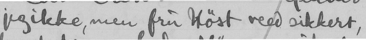jeg ikke, men fru Høst veed sikkert,
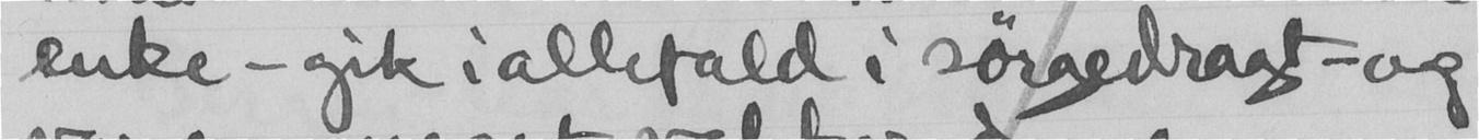enke - gik i allefald i sørgedragt - og
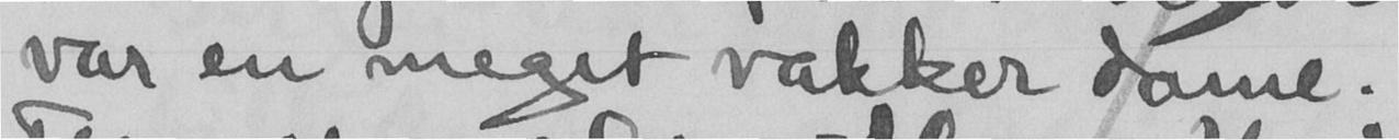var en meget vakker dame.
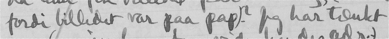fordi billidet var paa pap)? Jeg har tænkt
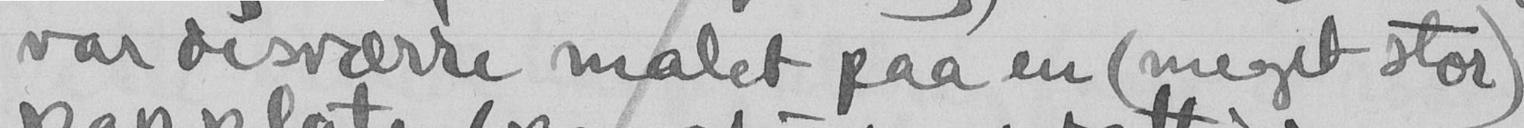var desværre malet paa en (meget stor)
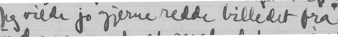Jeg vilde jo gjerne redde billedet fra
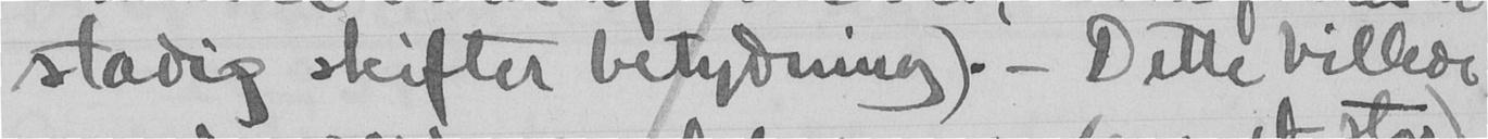stadig skifter betydning). - Dette billede
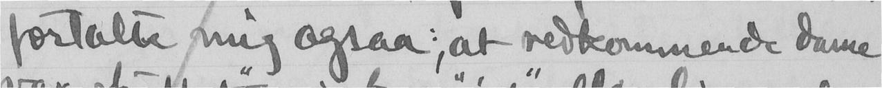fortalte mig ogsaa, at vedkommende dame
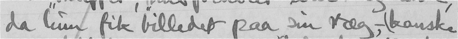da hun fik billedet paa sin væg- (kanske
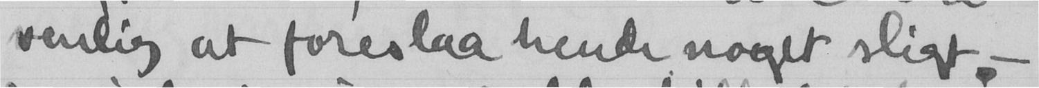venlig at foreslaa hende noget sligt. -
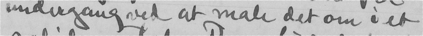undergang ved at male det om i et
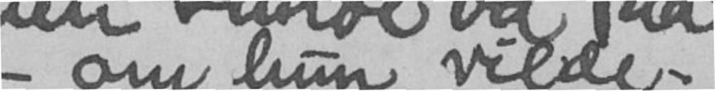- om hun vilde.
